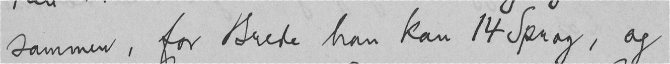sammen, for Brede han kan 14 Sprog, og
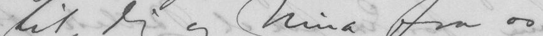til dig og Nina fra os
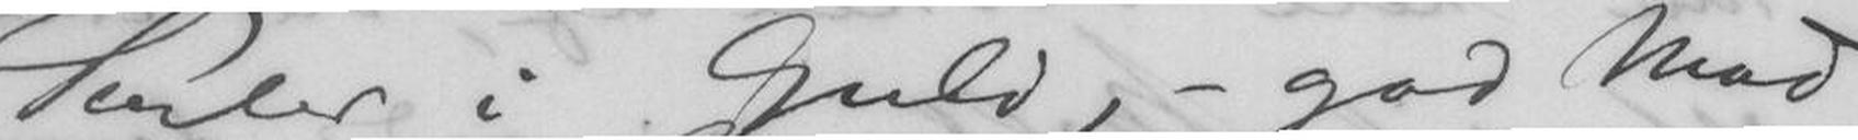Perler i Guld, - god Mad
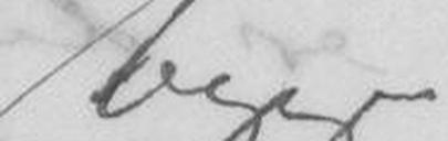begge!
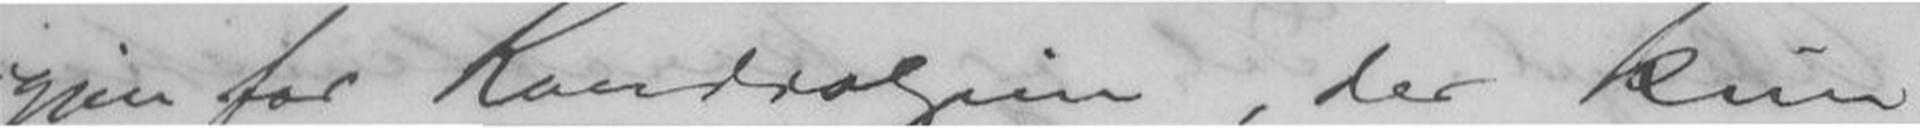igjen for Kardialgien, der kun
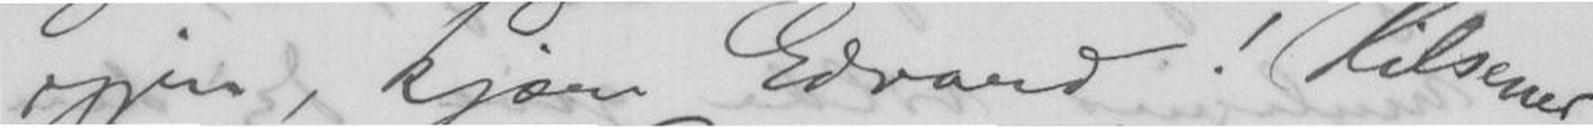igjen, kjære Edvard! Hilsener
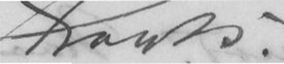Frants.
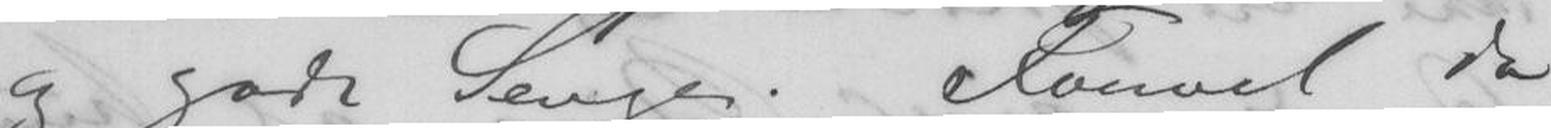og Gode Senge. Farvel da
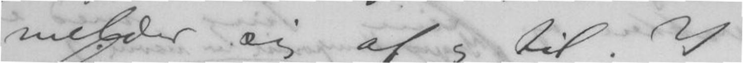melder sig af og til. I
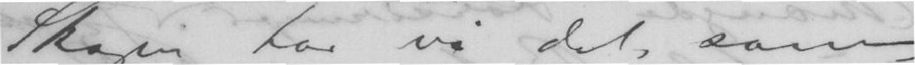Skogen har vi det, som
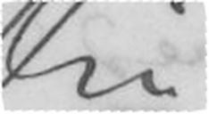Din
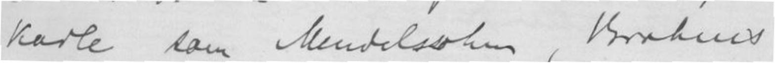karle som Mendelsohn, Brahms
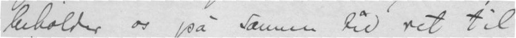beholder os på samme tid ret til
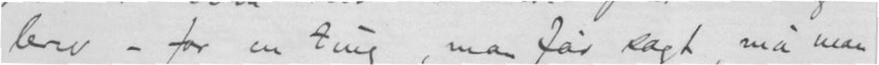brev - for en ting, man får sagt, må man
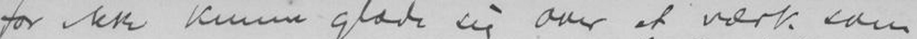for ikke kunne glæde mig over et værk som
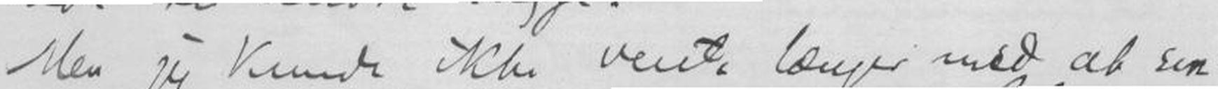Men jeg kunde ikke vente længer med at sen-
